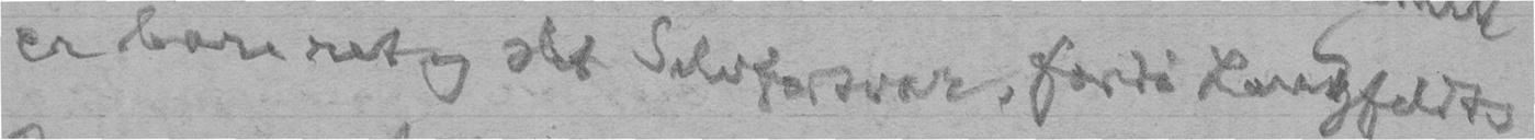er bare ret og slet Selvforsvar, fordi Langfeldts
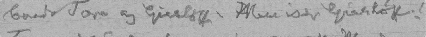baade Tore og Gierløff. Men især Giærløff!
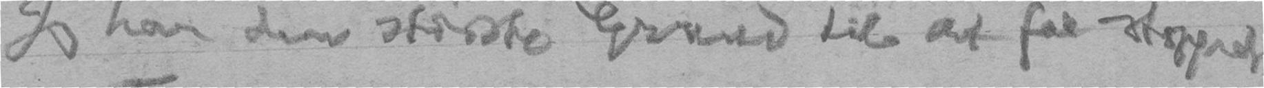Jeg har den største Grund til at faa stoppet
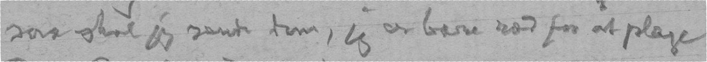saa skal jeg sende dem, jeg er bare ræd for at plage
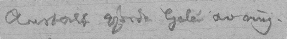Anstalt gjorde Gelé av mig.
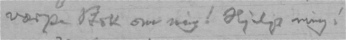værpe Bok om mig! Hjelp mig!
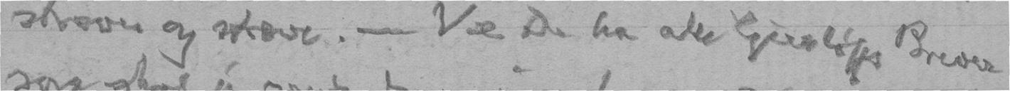stræve og stræve. - Vil De ha alle Gierløffs Brever
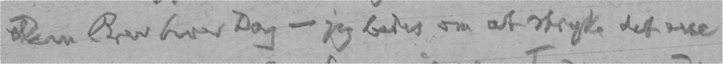Dem Brev hver Dag - jeg bedes om at stryke det ene
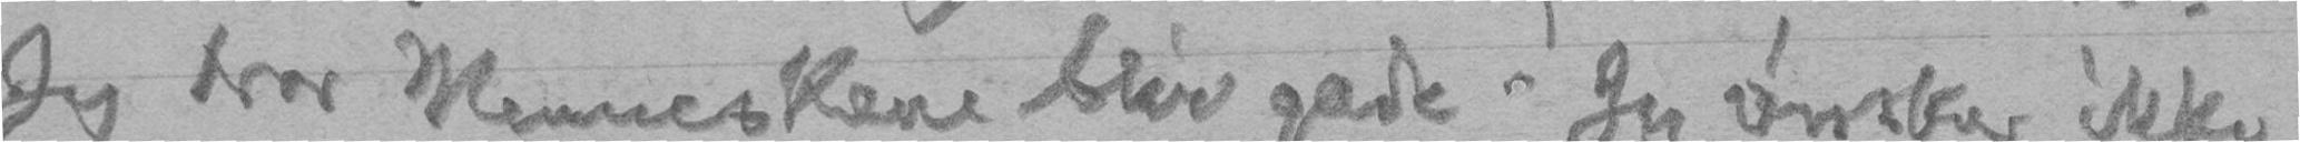Jeg tror Menneskene blir gale! Jeg ønsker ikke
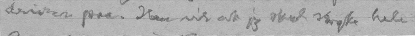driver paa. Han vil at jeg skal stryke hele
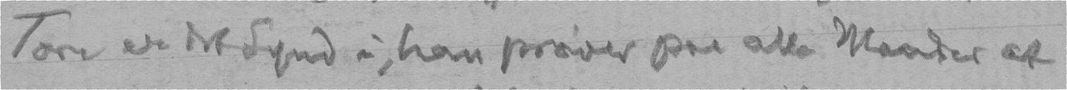Tore er det Synd i, han prøver paa alle Maader at
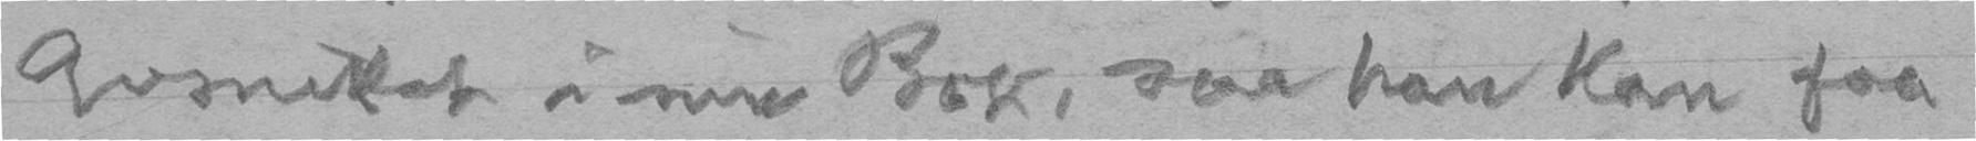Avsnittet i min Bok, saa han kan faa
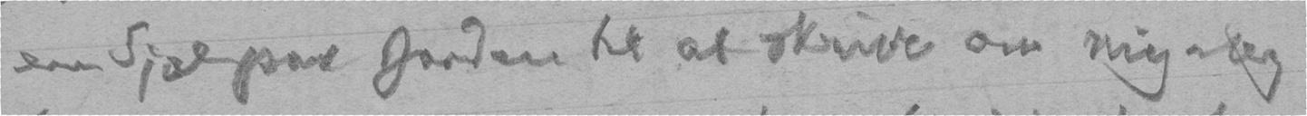en Sjæl paa Jorden til at skrive om mig, og
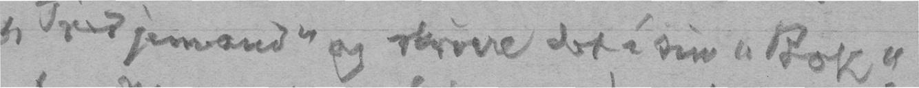"Tredjemand" og skrive det i sin "Bok".
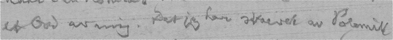et Ord av mig. Det jeg har skrevet av Polemik
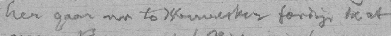her gaar nu to Mennesker færdige til at
